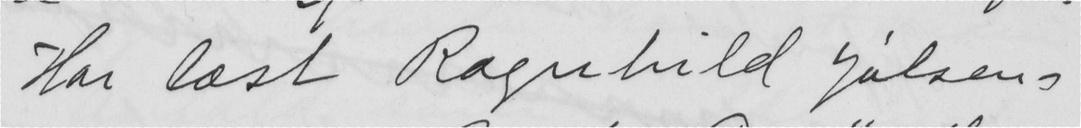Har læst Ragnhild Jølsens
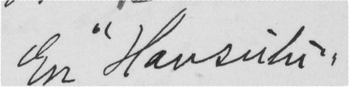En "Havsulu"
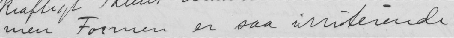men Formen er saa irriterende
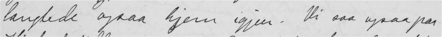langtede ogsaa hjem igjen. Vi saa ogsaa paa
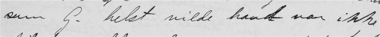som G. helst vilde havd var ikke
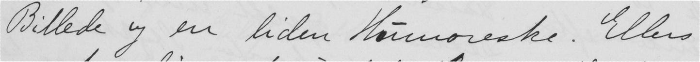Billede og en liden Humoreske. Ellers
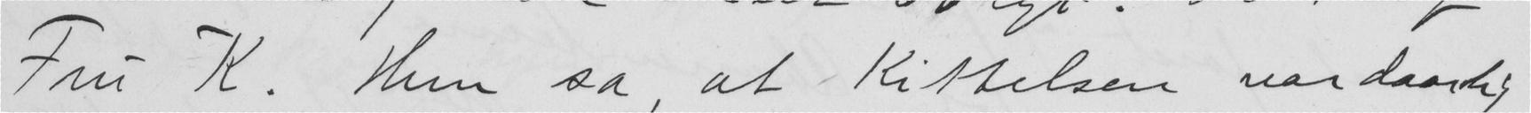Fru K. Hun sa at Kittelsen var daarlig
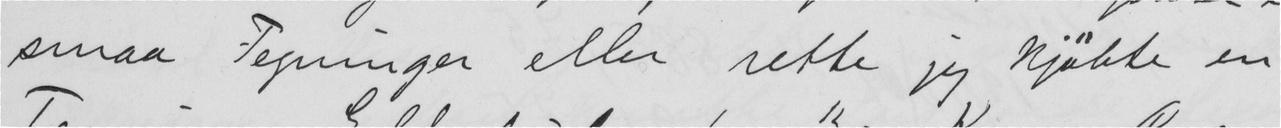smaa Tegninger eller rette jeg kjøbte en
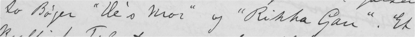to Bøger "Ve's Mor" og "Rikka Gan". Et
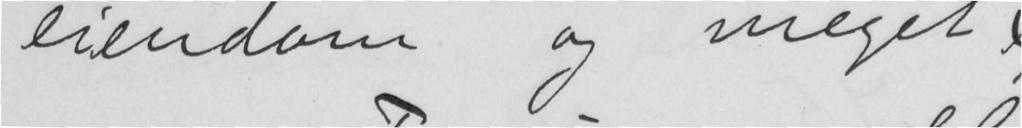eiendom og meget
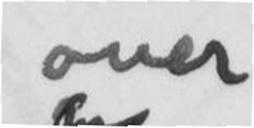over
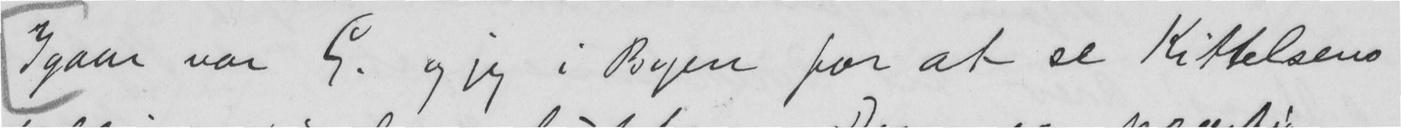Igaar var G. og jeg i Byen for at se Kittelsens
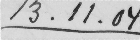13.11.04
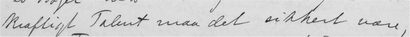kraftigt Talent maa det sikkert være,
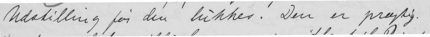Udstilling før den lukkes. Den er prægtig.
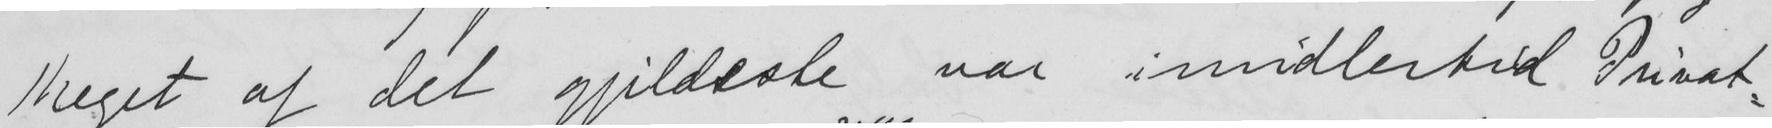Meget af det gjildeste var imidlertid Privat-
